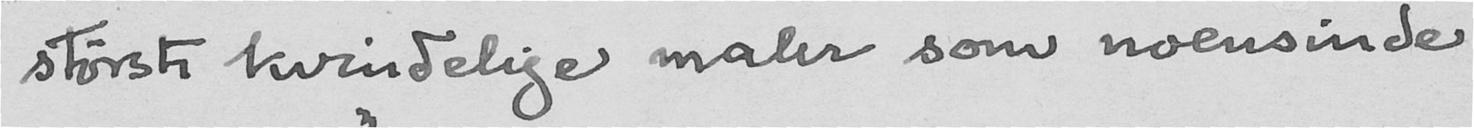største kvindelige maler som noensinde
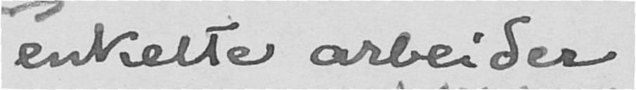enkelte arbeider,
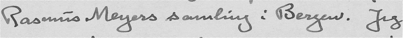Rasmus Meyers samling i Bergen. Jeg
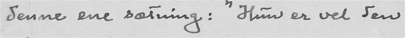denne ene sætning: "Hun er vel den
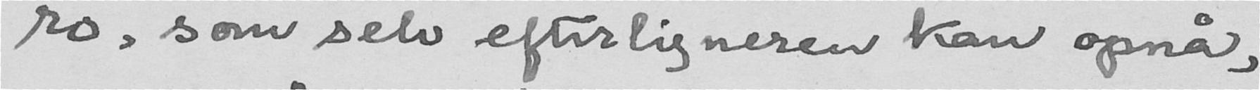ro, som selv efterligneren kan opnå,
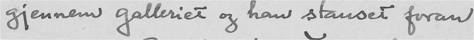gjennem galleriet og han stanset foran
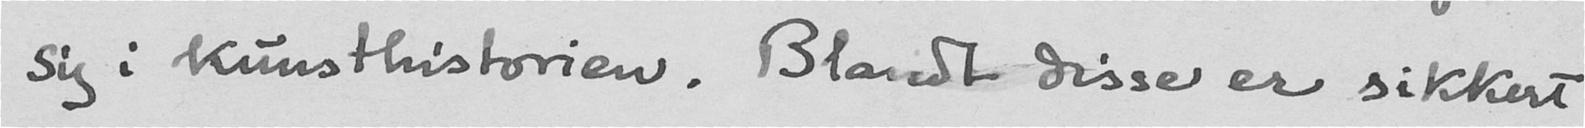sig i kunsthistorien. Blandt disse er sikkert
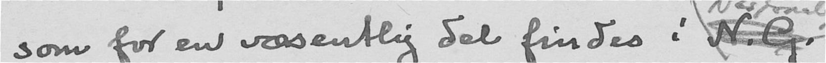som for en væsentlig del findes i N.G.
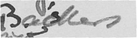Backers
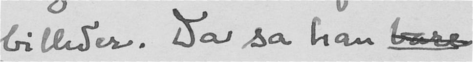billeder. Da så han bare
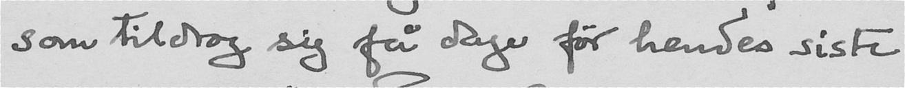som tildrog sig få dage før hendes siste
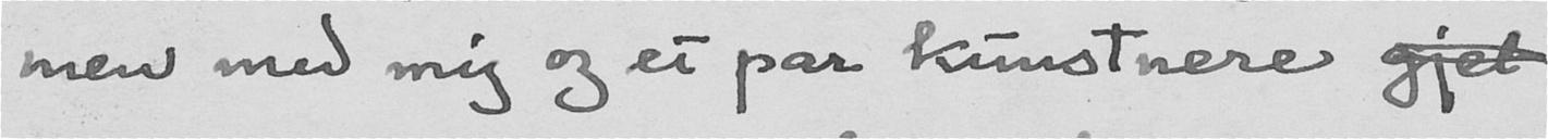men med mig og et par kunstnere gjel
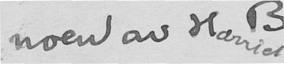noen av Harriet
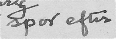spor efter
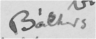Backers
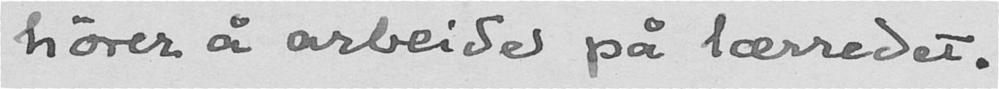hører å arbeide på lærredet.
